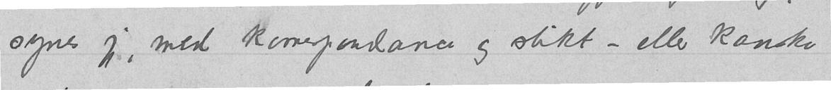synes jeg, med korrespondance og slikt - eller kanske
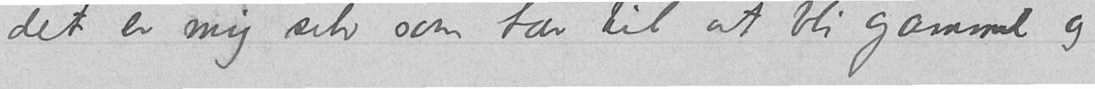det er mig selv som tar til at bli gammel og
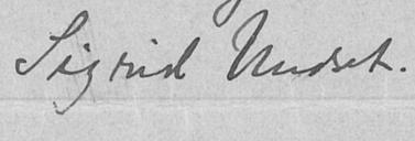Sigrid Undset.
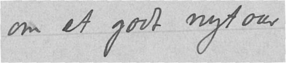om et godt nytaar
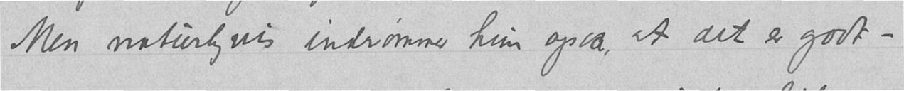Men naturligvis indrømmer hun ogsaa, at det er godt –
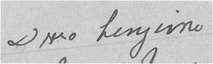Deres hengivne
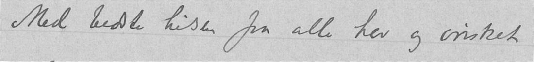Med bedste hilsen fra alle her og ønsket
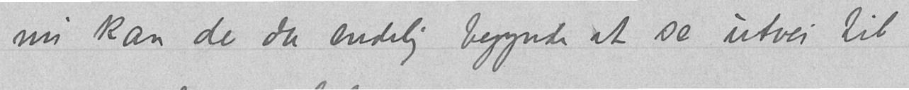nu kan de da endelig begynde at se utvei til
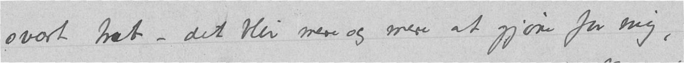svært træt – det blir mere og mere at gjøre for mig,
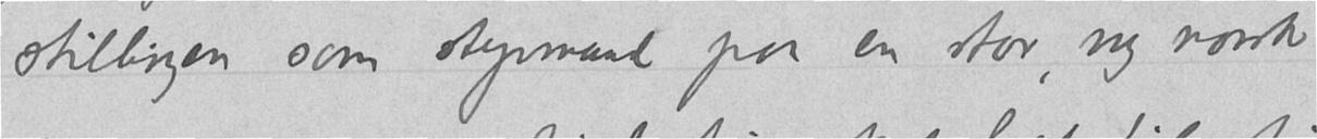stillingen som styrmand paa en stor, ny norsk
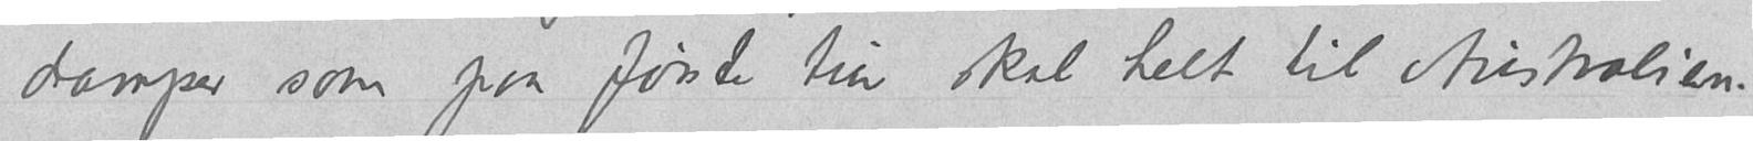damper som paa første tur skal helt til Australien.
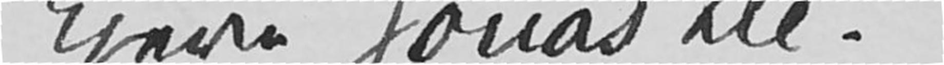Kjere Jonas Lie!
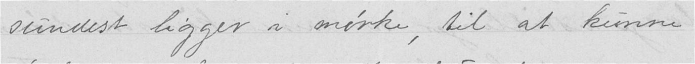sundest ligger i mørke, til at kunne
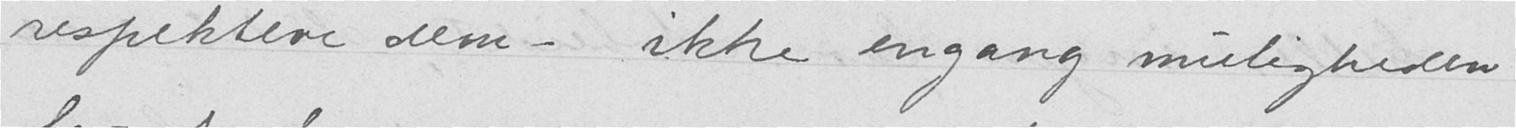respektere dem - ikke engang muligheden
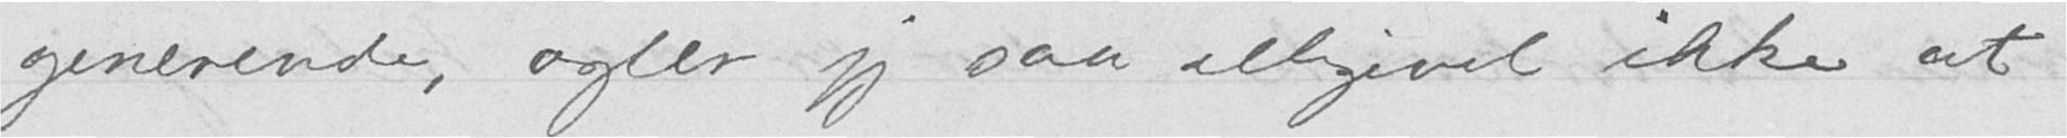generende, agter jeg saa alligevel ikke at
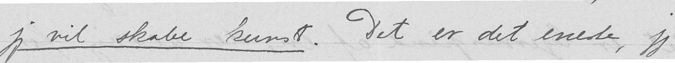jeg vil skabe kunst. Det er det eneste, jeg
